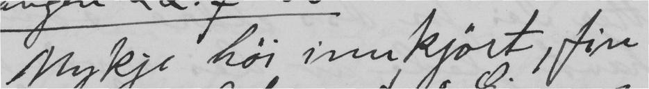Mykje höi innkjört, fin
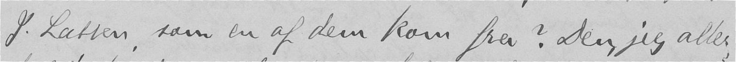I. Lassen, som en af dem kom fra? Den, jeg aller-
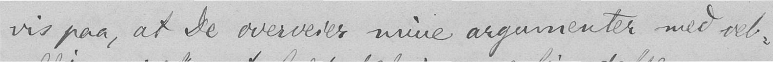vis paa, at De overveier mine argumenter med vel-
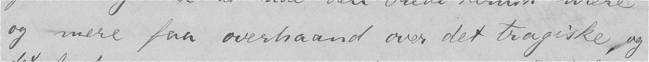og mere faa overhaand over det tragiske, og
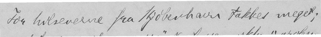For hilsenerne fra Kjøbenhavn takkes meget;
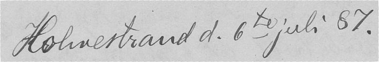Holmestrand d. 6te juli 87.
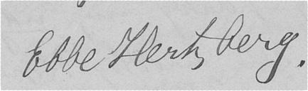Ebbe Hertzberg.
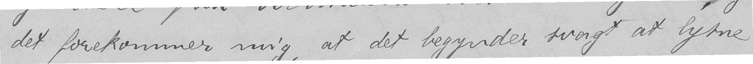det forekommer mig, at det begynder svagt at lysne
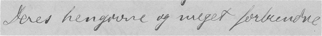Deres hengivne og meget forbundne
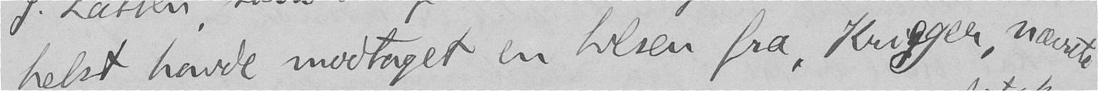helst havde modtaget en hilsen fra, Krigger, nævnte
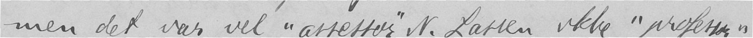men det var vel "assessor" N. Lassen, ikke "professor"
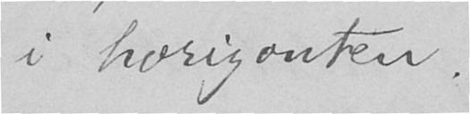i horizonten.
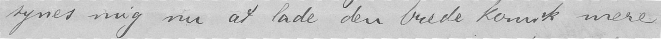synes mig nu at lade den brede komik mere
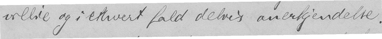villie og i ethvert fald delvis anerkjendelse.
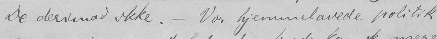De derimod ikke. - Vor hjemmelavede politik
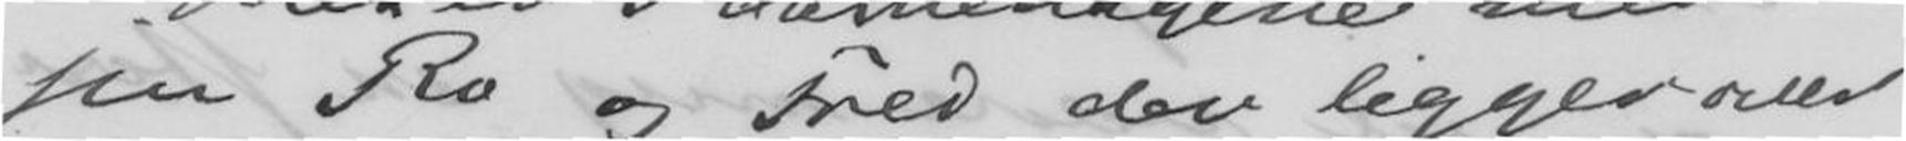sin Ro og Fred der ligger over
